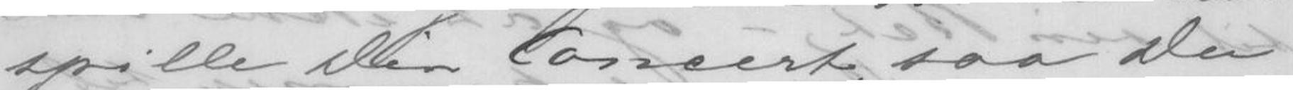spille din Concert, saa Du
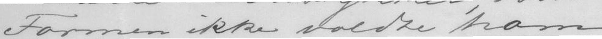Formen ikke voldte ham
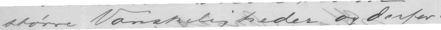større Vanskeligheder og derfor
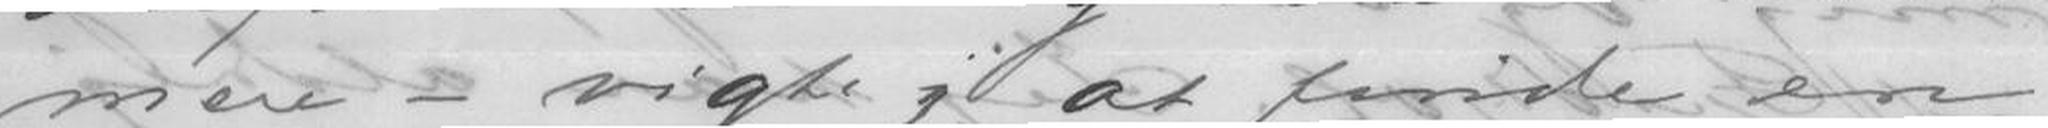mere - vigtig at finde en
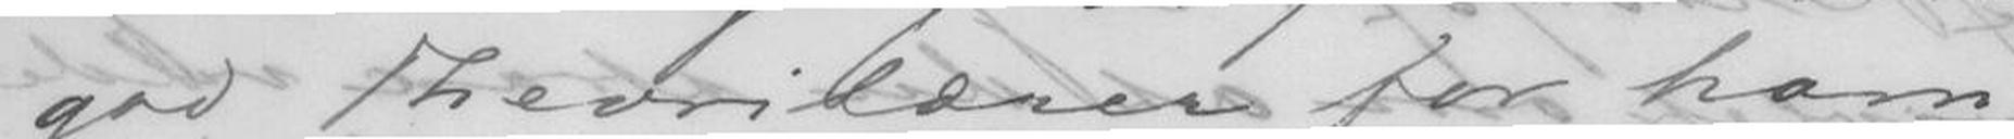god Theorilærer for ham,
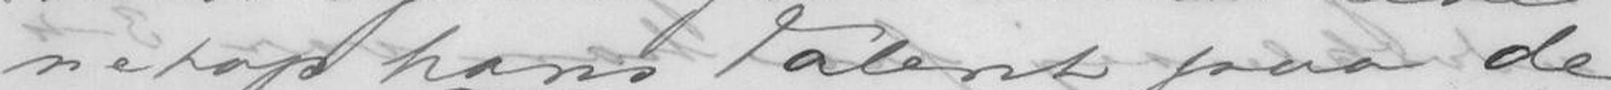netop hans Talent paa de
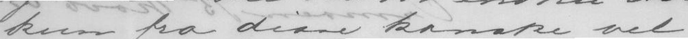kun fra disse kanske vel
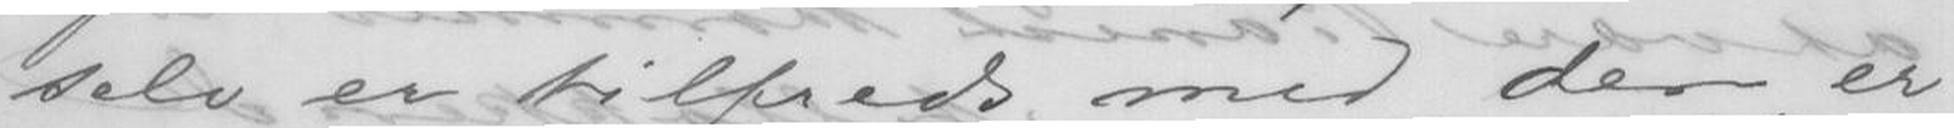selv er tilfreds med den, er
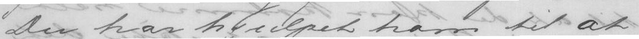Du har hjulpet ham til at
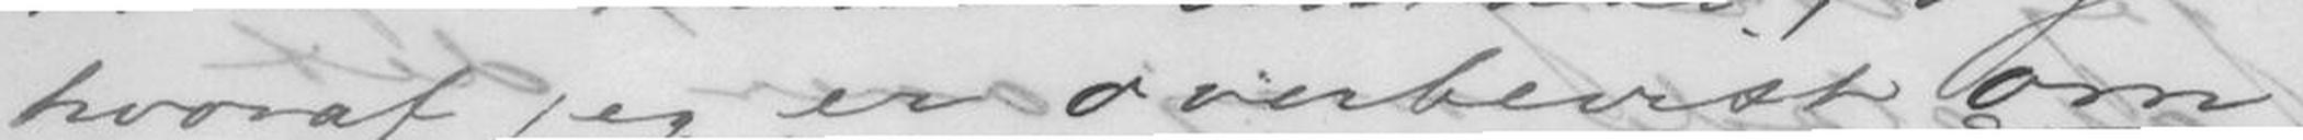hvoraf jeg er overbevist om
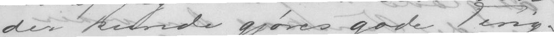der kunde gjøres gode Ting;
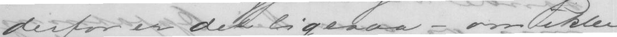derfor er det ligesaa - om ikke
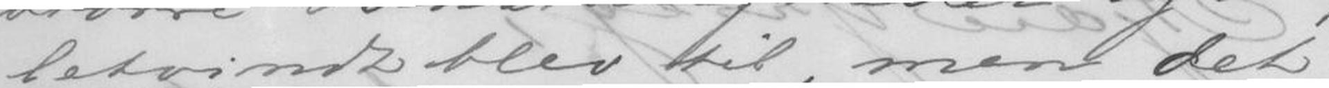letvindt blev til, men det
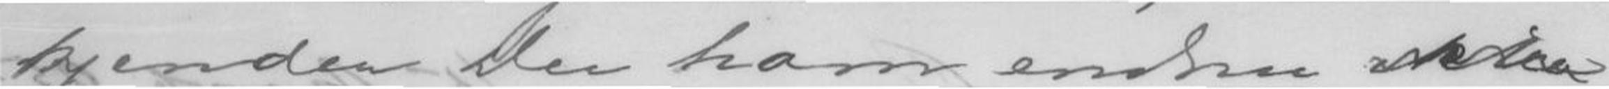kjender Du ham endnu
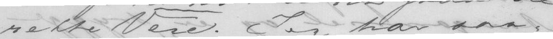rette Veie. Jeg har saa
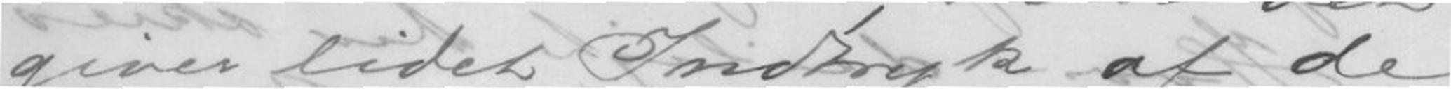giver lidet Indtryk af de
